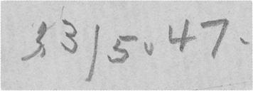13/5. 47.
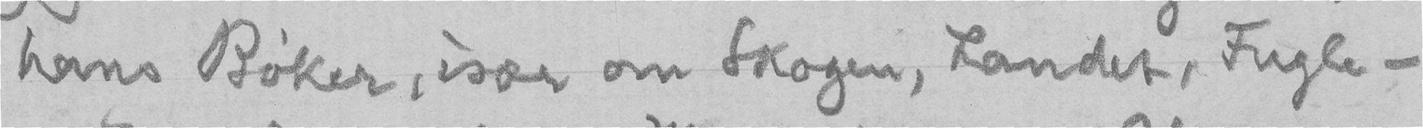hans Bøker, især om Skogen, Landet, Fugle-
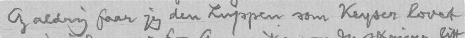Og aldrig faar jeg den Luppen som Keyser lovet
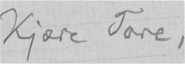Kjære Tore,
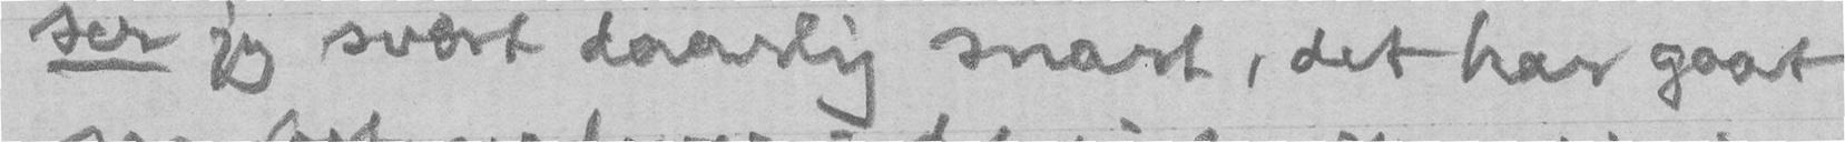ser jeg svært daarlig snart, det har gaat
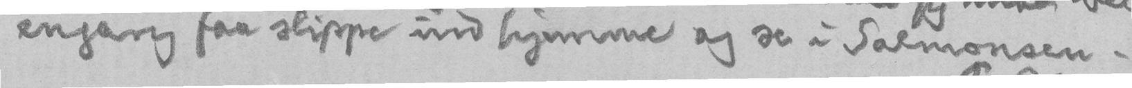engang faa slippe ind hjemme og se i Salmonsen.
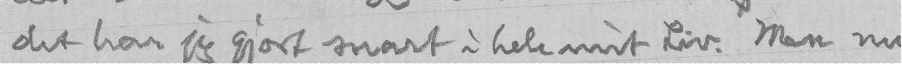det har jeg gjort snart i hele mit Liv. Men nu
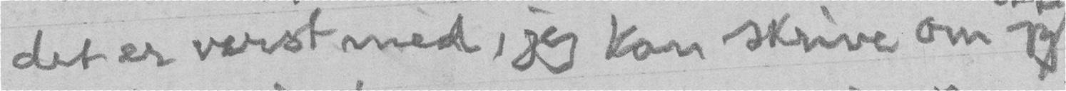det er verst med, jeg kan skrive om jeg
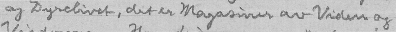og Dyrelivet, det er Magasiner av Viden og
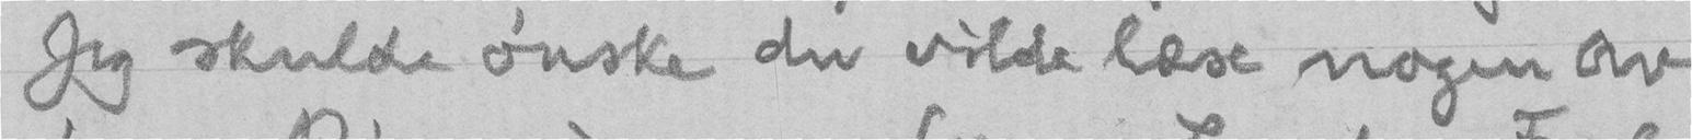Jeg skulde ønske du vilde læse nogen av
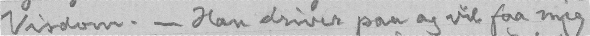Visdom. - Han driver paa og vil faa mig
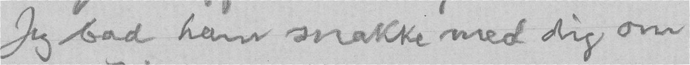Jeg bad ham snakke med dig om
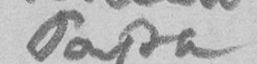Papa
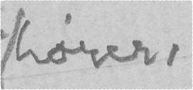hører,
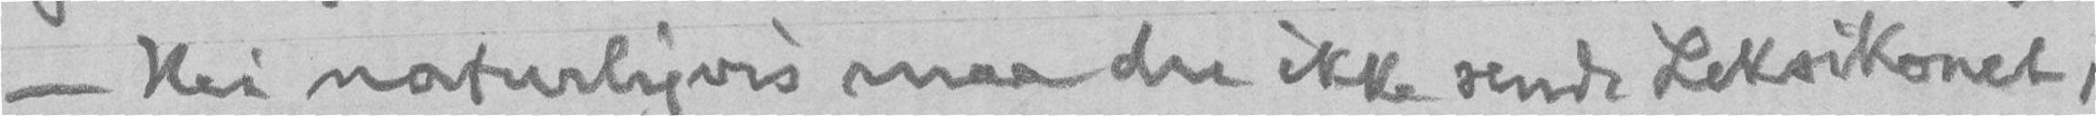- Nei naturligvis maa du ikke sende Leksikonet,
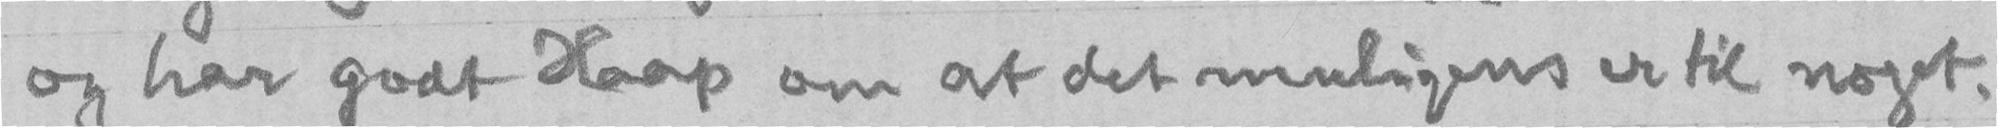og har godt Haap om at det muligens er til noget.
